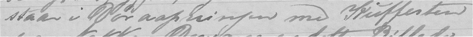staar i Døraapningen med Kufferten
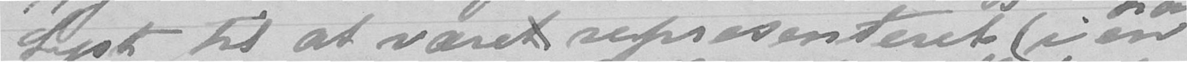Lyst til at været representeret (i en
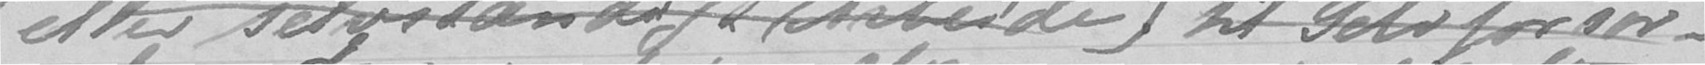eller selvstændigt Arbeide) til Selvforsør-
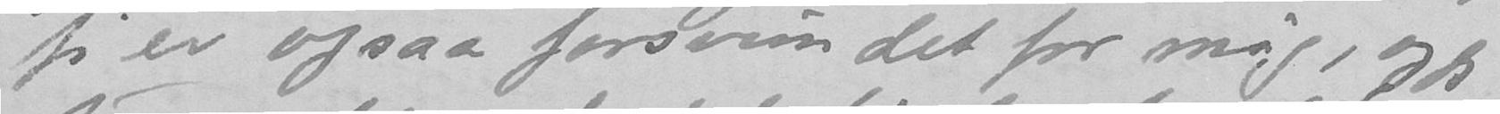fi er ogsaa forsvundet for mig, og jeg
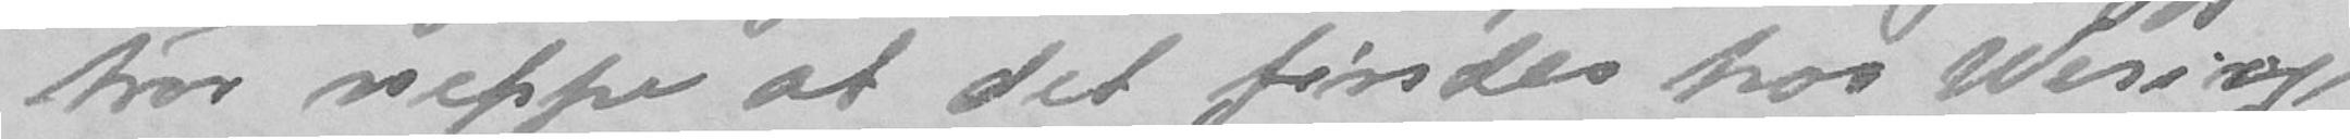tror neppe at det findes hos Wering,
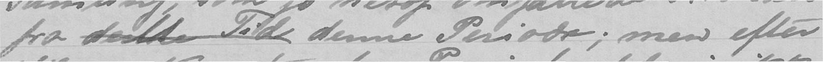fra dette Tid denne Periode; men efter
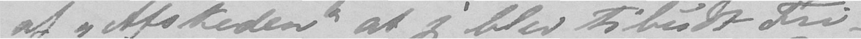af "Afskeden" at jeg blev tibudt Fri-
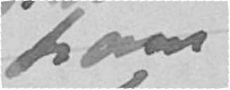hans
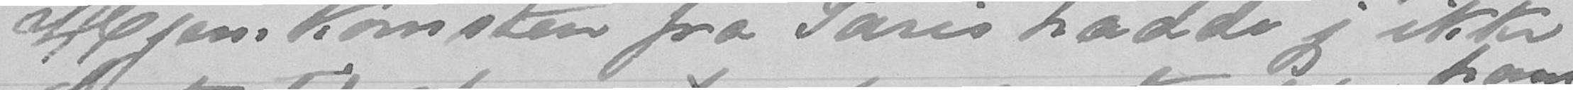Hjem-komsten fra Paris hadde jeg ikke
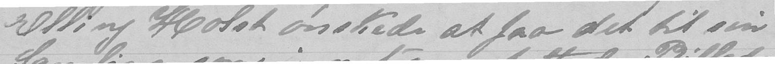Elling Holst ønskede at faa det til sin
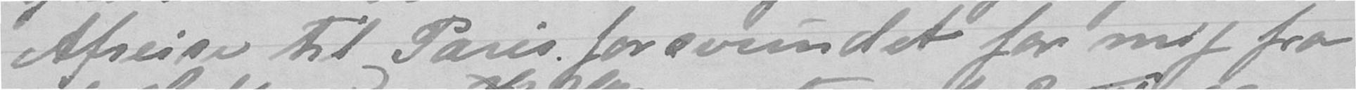Afreise til Paris forsvundet for mig fra
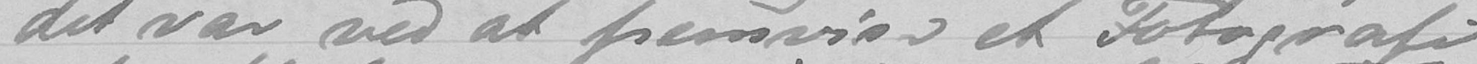det var ved at fremvise et Fotografi
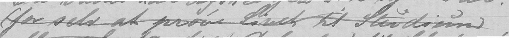(for selv at prøve Livet til Studium,
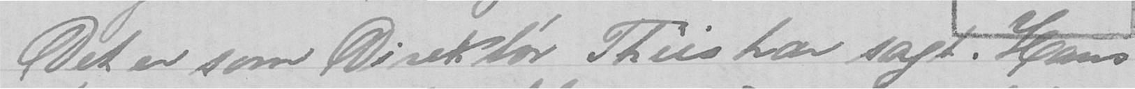Det er som Direktør Thiis har sagt. Hans
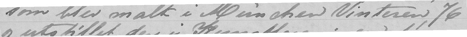som blev malt i München Vinteren 76
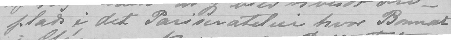plads i det Pariseratelier hvor Bomat
